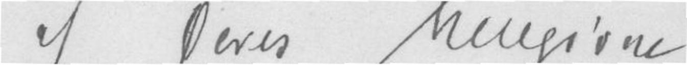af Deres hengivne
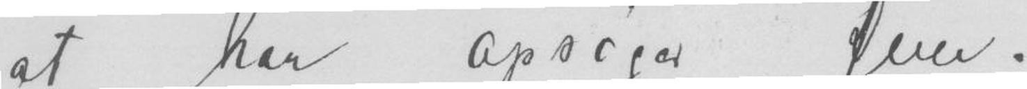at han opsøger Dem.
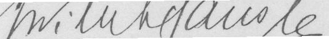Wilhelm Hansen
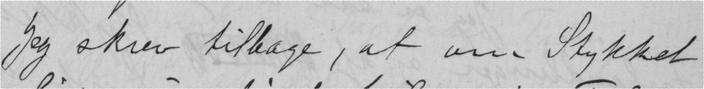Jeg skrev tilbage, at om Stykket
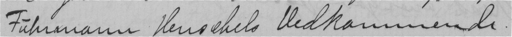Fuhrmann Henschels Vedkommende.
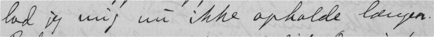lod jeg mig nu ikke opholde længer.
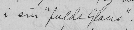i sin "fulde Glans".
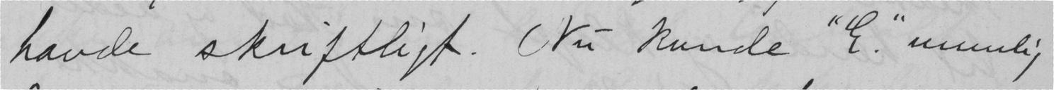havde skriftligt. Nu kunde "E". umulig
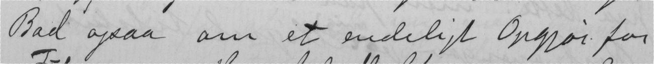Bad ogsaa om et endeligt Opgjør for
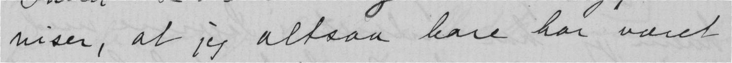viser, at jeg altsaa bare har været
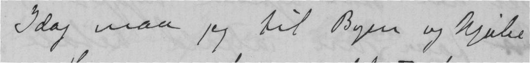Idag maa jeg til Byen og kjøbe
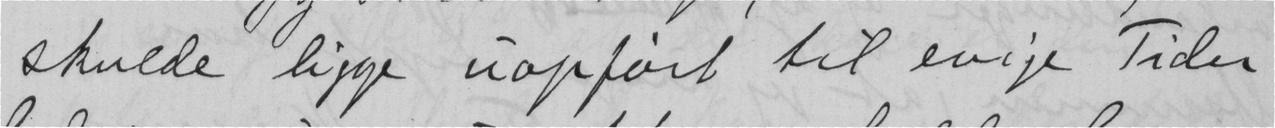skulde ligge uopført til evige Tider
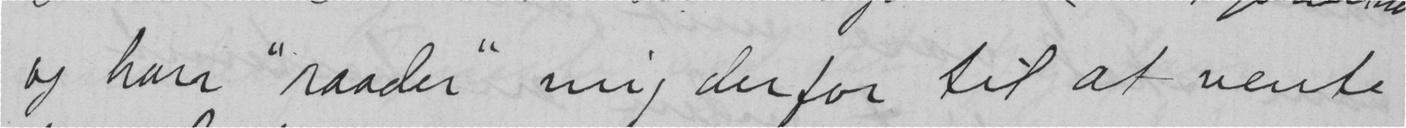og han "raader" mig derfor til at vente
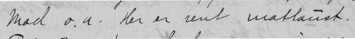mad o. a. Her er rent matlaust.
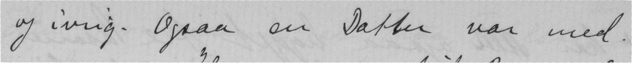og ivrig. Ogsaa en Datter var med.
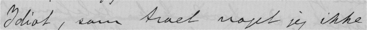Idiot, som troet noget jeg ikke
